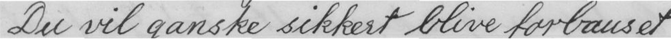Du vil ganske sikkert blive forbauset
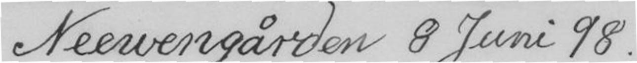Neewergården 8 Juni 98.
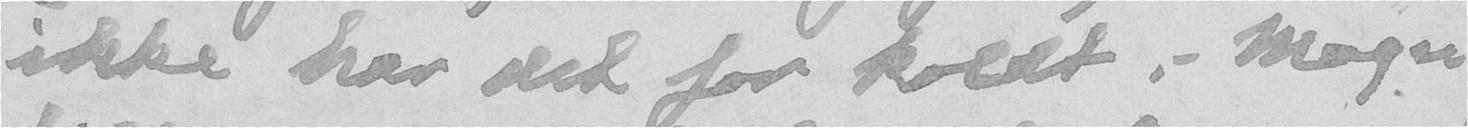ikke har det for koldt, - Magn-
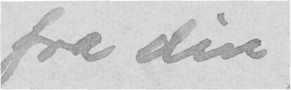fra din
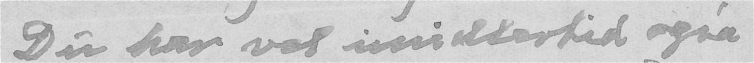Du har vel imidtertid også
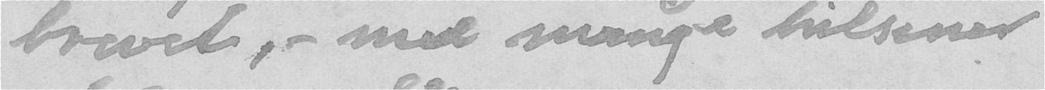brevet, - med mange hilsener
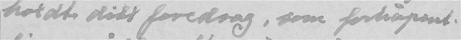holdt ditt foredrag, som forhåpent-
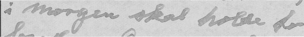i morgen skal holde to forskjellige
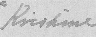Kristine
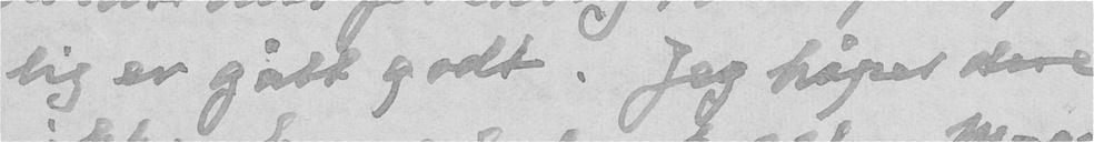lig er gått godt. Jeg håper dere
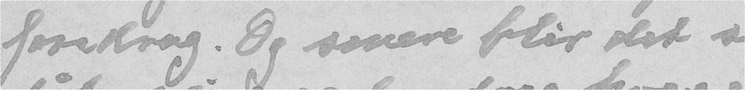foredrag. Og senere blir det så
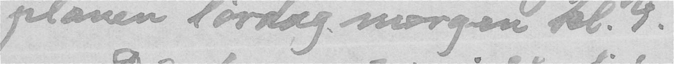planen lørdag morgen kl. 9.
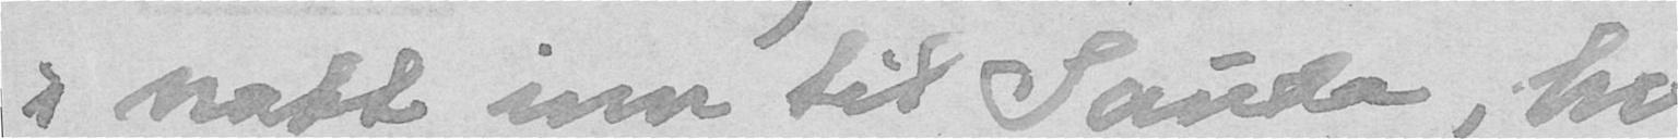i natt inn til Sauda, hvor jeg
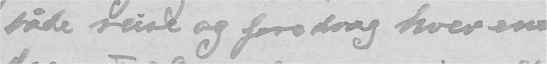både reise og foredrag hver eneste
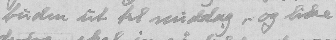buden ut til middag, og like
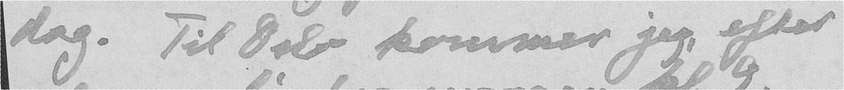dag. Til Oslo kommer jeg, efter
